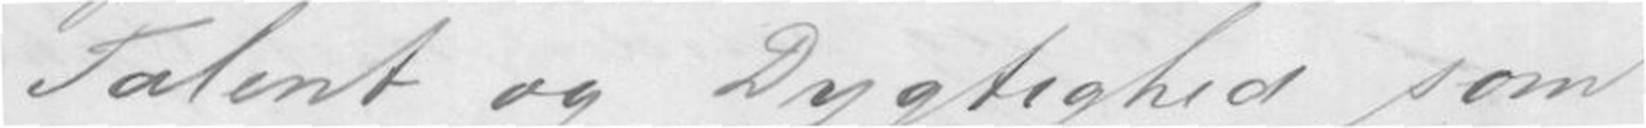Talent og Dygtighed som
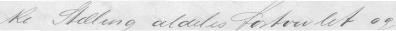ke Stilling aldeles Fortvivlet og
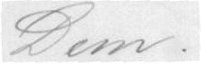Dem.
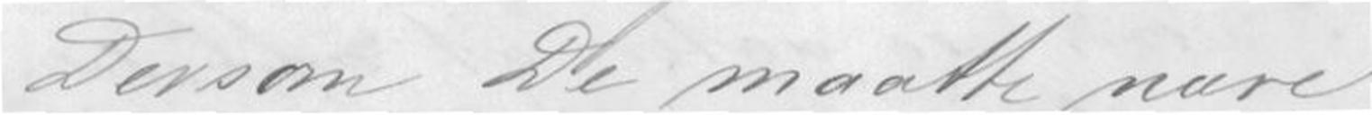Dersom De maatte være
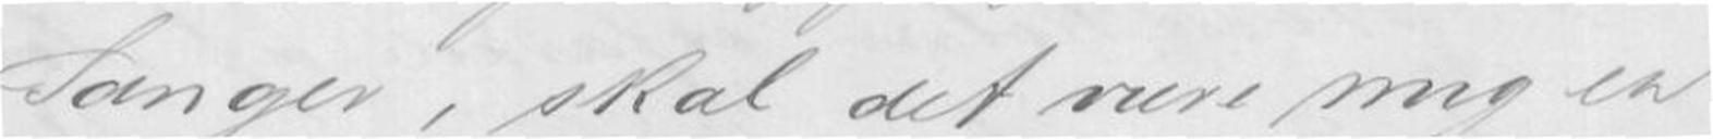Sanger, skal det være mig en
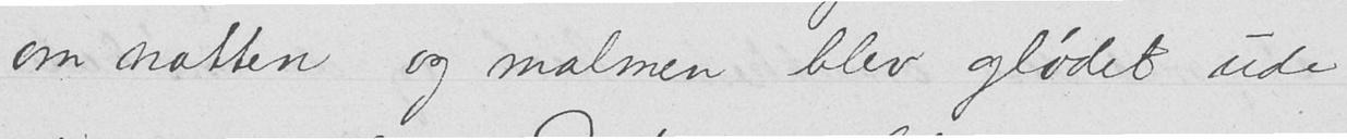om natten og malmen blev glødet ude
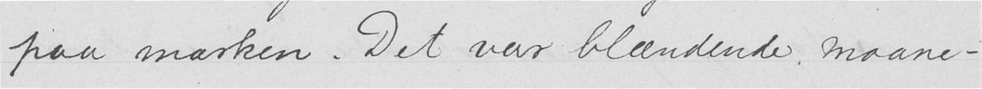paa marken. Det var blændende maane-
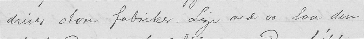driver store fabriker. Lige ved os laa den
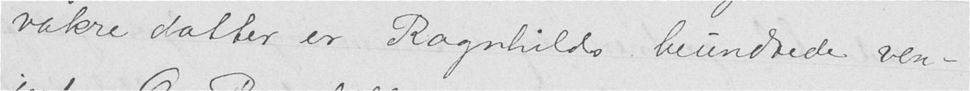vakre datter er Ragnhilds beundrede ven-
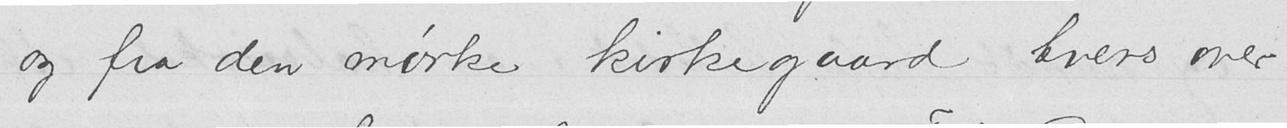og fra den mørke kirkegaard tvers over
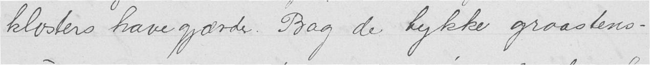klosters havegjærde. Bag de tykke graastens-
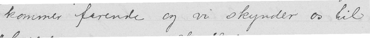kommer farende og vi skynder os til
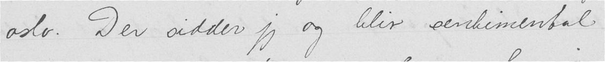Oslo. Der sidder jeg og blir sentimental
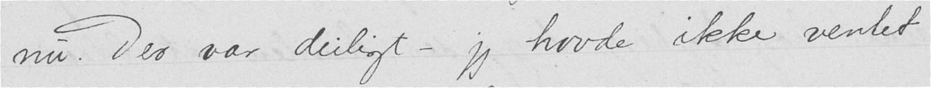nu. Der var deiligt — jeg havde ikke ventet
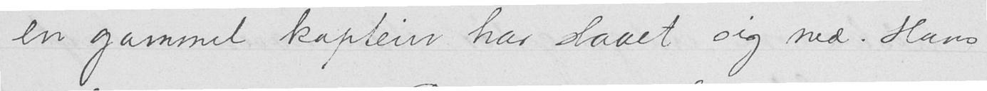en gammel kaptein har slaaet sig ned. Hans
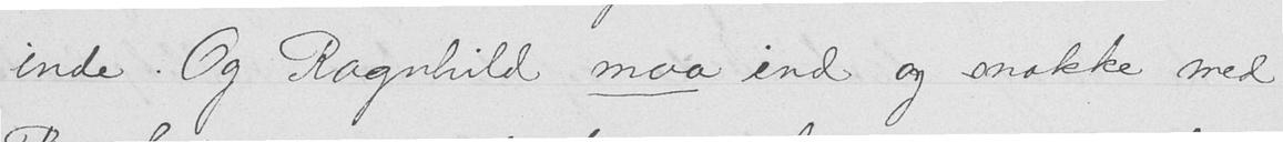inde. Og Ragnhild maa ind og snakke med
